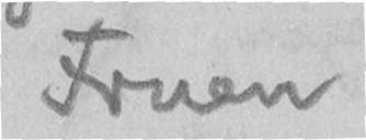Fruen
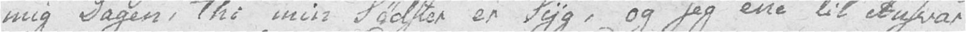mig Dagen, thi min Sødster er Syg, og jeg ene til Ansvar
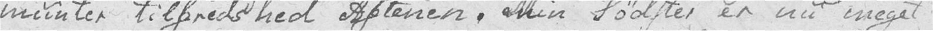munter tilfredshed Aftenen. Min Sødster er nu meget
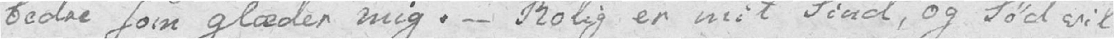bedre som glæder mig. – Rolig er mit Sind, og Sød vil
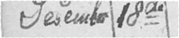Desember 18de
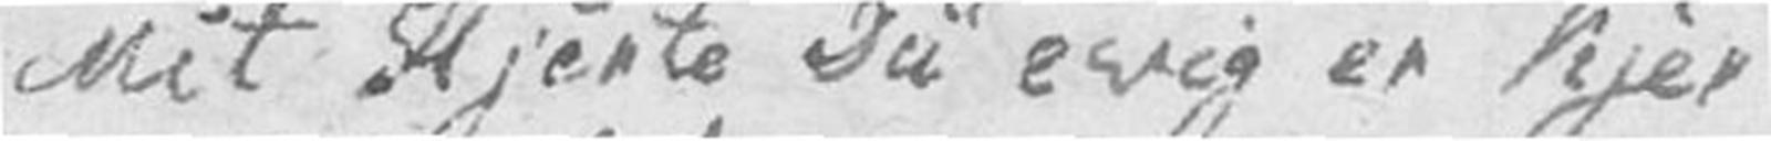Mit Hjerte Du evig er kjer
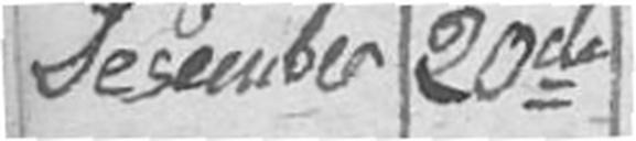Desember 20de
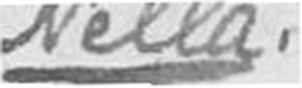Nella
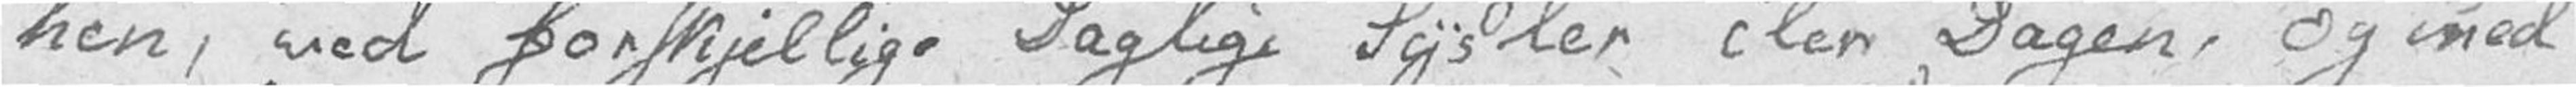hen, ved forskjellige Daglige Sysler iler Dagen, og med
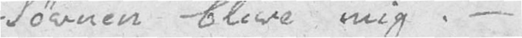Søvnen blive mig. –
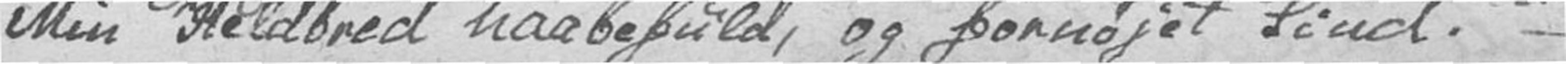Min Heldbred haabefuld, og fornøjet Sind. –
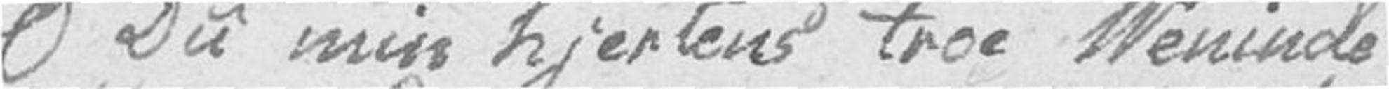O Du min hjertens troe Veninde
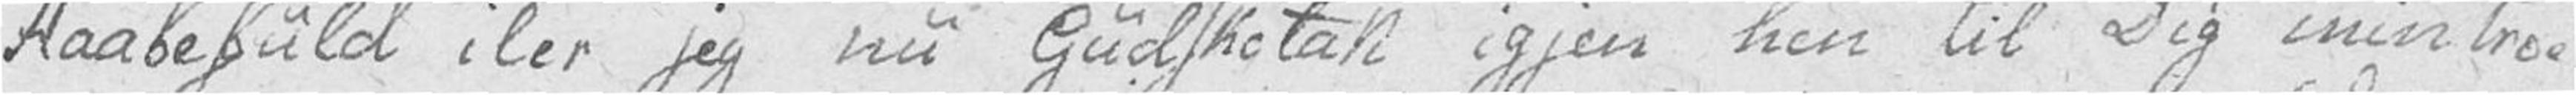Haabefuld iler jeg nu Gudsketak igjen hen til Dig min troe
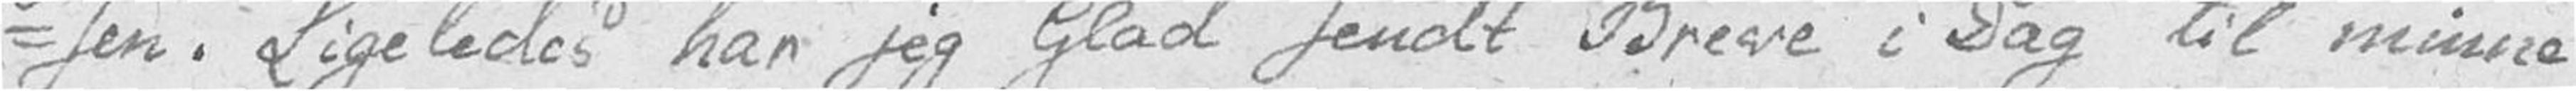-sen. Ligeledes har jeg Glad sendt Breve i Dag til minne
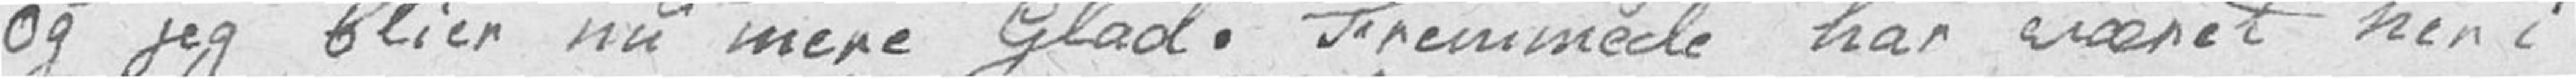og jeg blier nu mere Glad. Fremmede har været her i
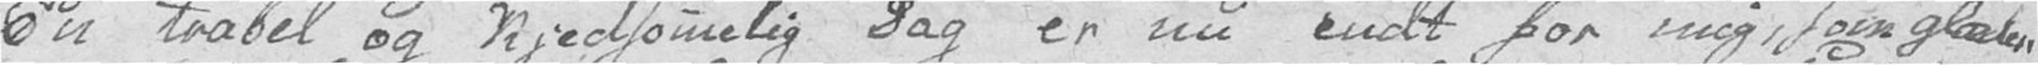En trabel og Kjedsomelig Dag er nu endt for mig, som glæder
